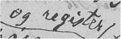og register
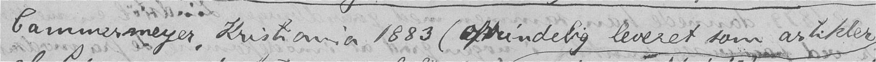Cammermeyer, Kristiania 1883 (oprindelig leveret som artikler
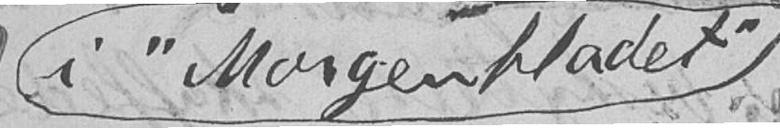i "Morgenbladet"
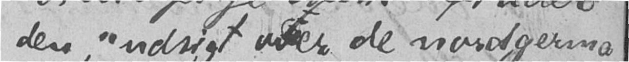den "udsigt over de nordgerma-
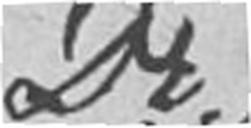Dr.
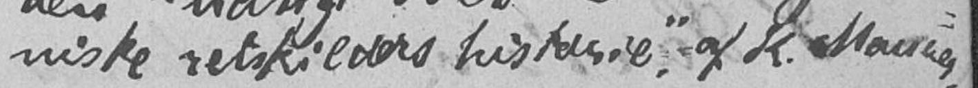niske retskilders historie", af K. Maurer,
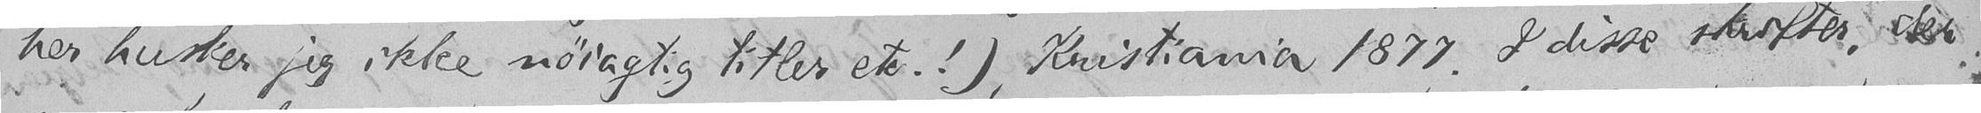her husker jeg ikke nøiagtig titler etc.!), Kristiania 1877. I disse skrifter, der
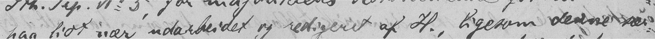paa lidt nær udarbeidet og redigeret af H., ligesom denne sær-
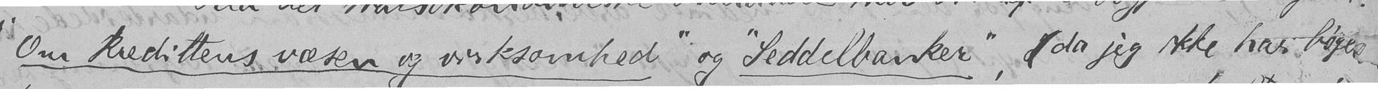"Om kredittens væsen og virksomhed" og "Seddelbanker", (da jeg ikke har bøger
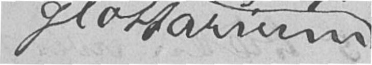glossarium
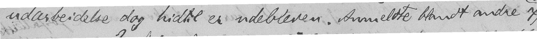udarbeidelse dog hidtil er udebleven. Anmeldte blandt andre af
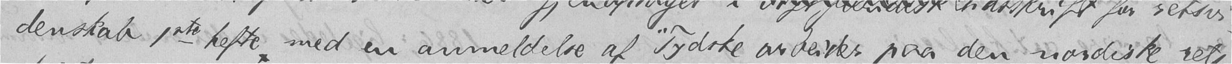denskab 1ste hefte med en anmeldelse af "Tydske arbeider paa den nordiske rets-
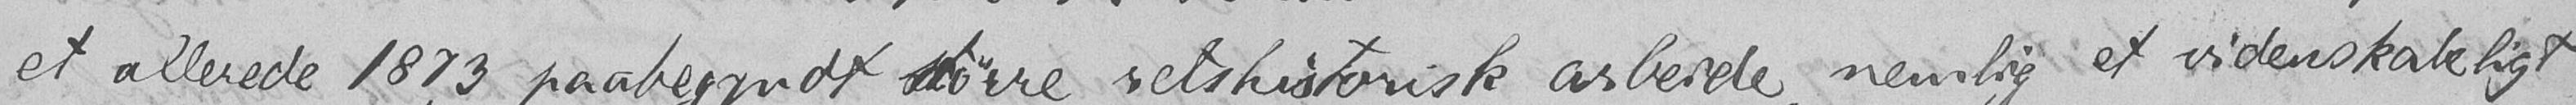et allerede 1873 paabegyndt større, retshistorisk arbeide, nemlig et videnskabeligt
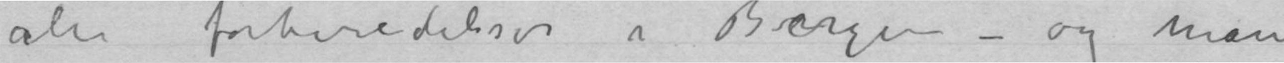alle forberedelser i Bergen – og man
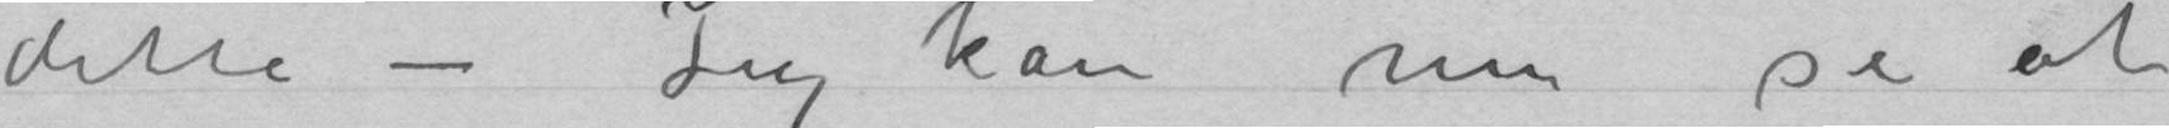dette – Jeg kan nu se at
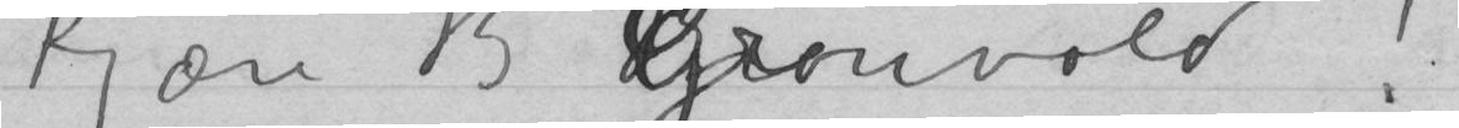Kjære B Grønvold!
